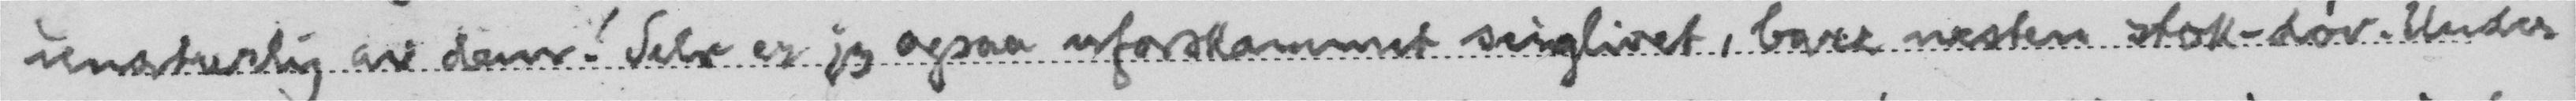unaturlig av dem! Selv er jeg ogsaa uforskammet seiglivet, bare nesten stok-døv. Under
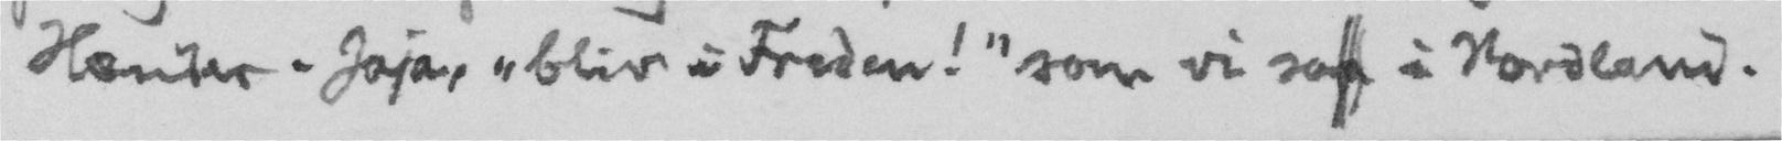Hænder. Jaja, "bliv i Freden!" som vi saa i Nordland.
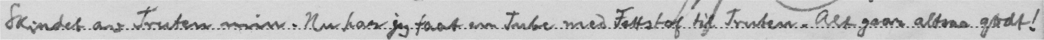Skindet av Truten min. Nu har jeg faat en Tube med Fettstof til Truten. Alt gaar altsaa godt!
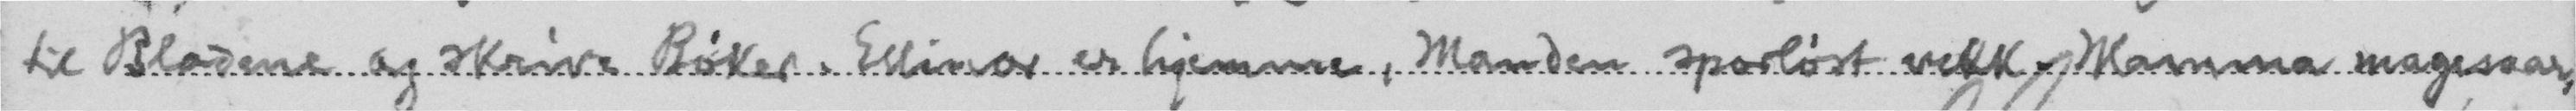til Bladene og skrive Bøker. Ellinor er hjemme, Manden sporløst vekk. Mamma magesaar,
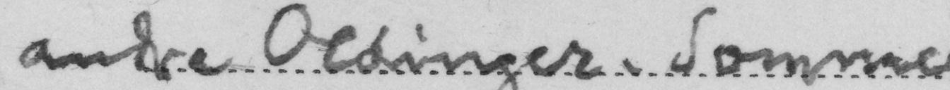andre Oldinger. Somme
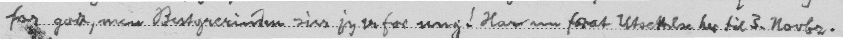for godt, men Bestyrerinden sier jeg er for ung! Har nu faat Utsettelse her til 3. Novbr.
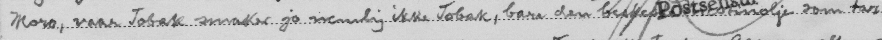Moro, vaar Tobak smaker jo nemlig ikke Tobak, bare den beskeste Nicotinolje som tar
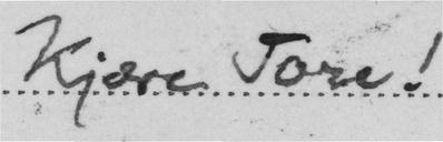Kjære Tore!
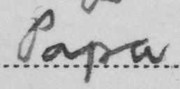Papa
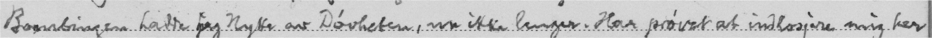Bombingen hadde jeg Nytte av Døvheten, nu ikke lenger. Har prøvet at indlosjere mig her
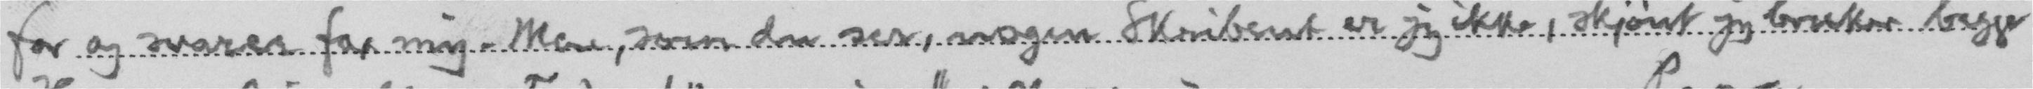for og svarer for mig. Men, som du ser, nogen Skribent er jeg ikke, skjønt jeg bruker begge
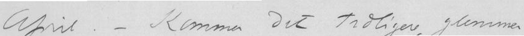April. - Kommer det tidligere, glemmer
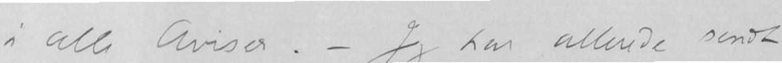i alle Aviser. - Jeg har allerede sendt
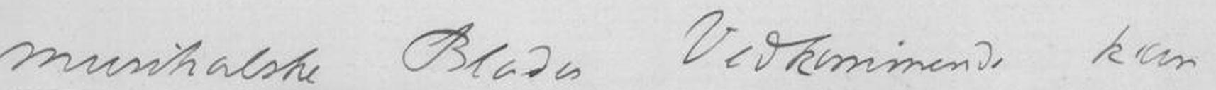musikalske Blades Vedkommende kan
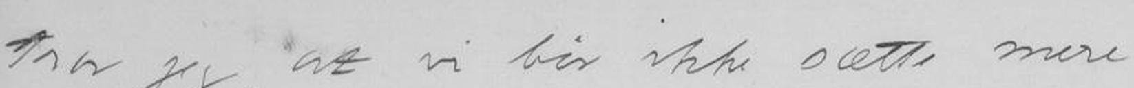tror jeg, at vi bør ikke sætte mere
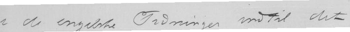i de engelske Tidninger indtil det
